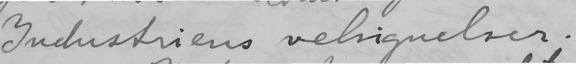Industriens velsignelser.
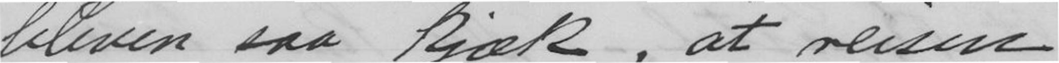bleven saa kjæk, at reisen
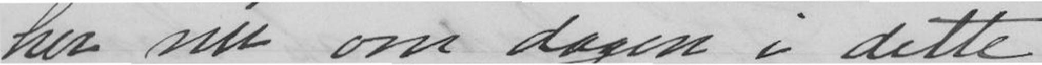her nu om dagen i dette
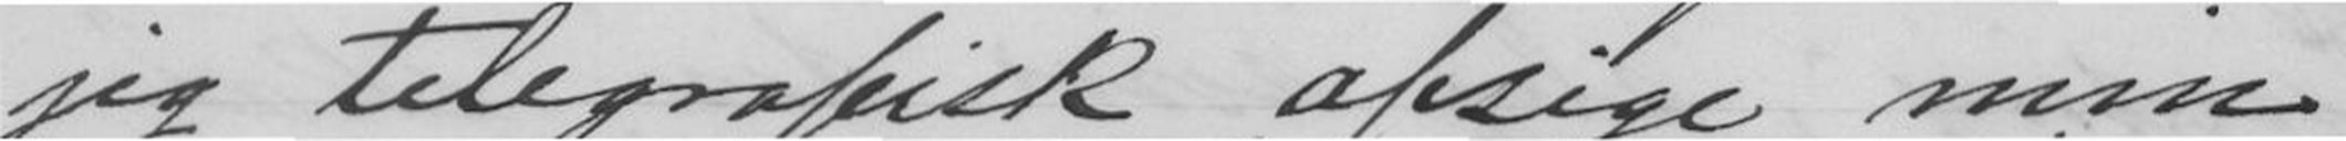jeg telegrafisk afsige min
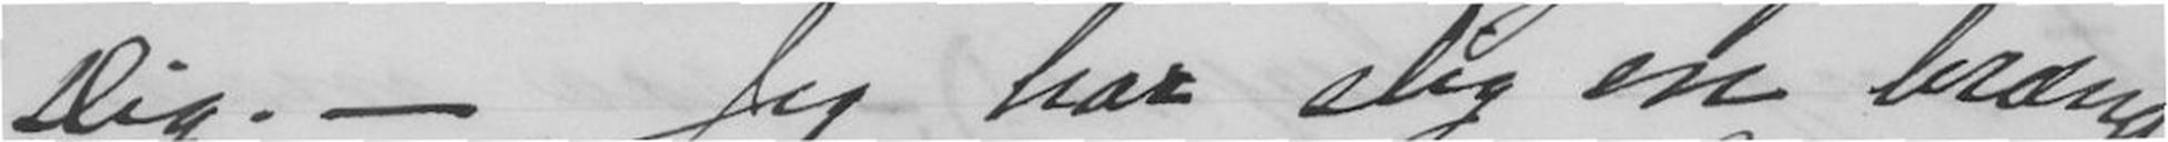Dig. - Jeg har slig en brænd-
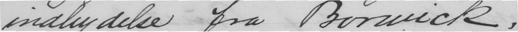indbydelse fra Borwick,
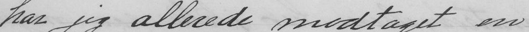har jeg allerede modtaget en
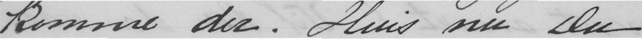komme der. Hvis nu Du
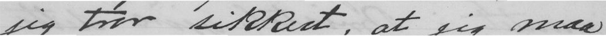jeg tror sikkert, at jeg maa
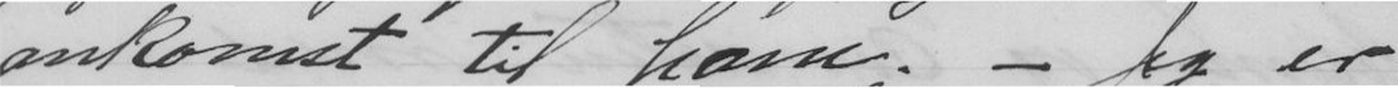ankomst til ham. - Jeg er
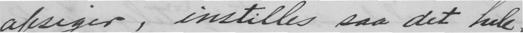afsiger, instilles saa det hele
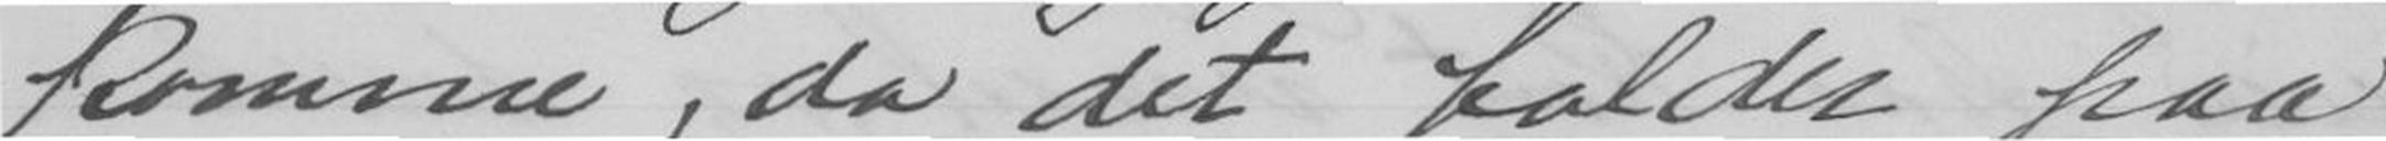komme, da det falder paa
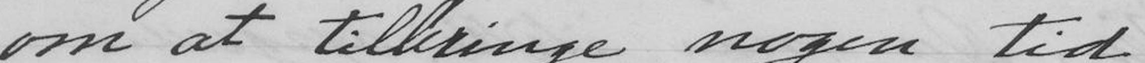om at tilbringe nogen tid
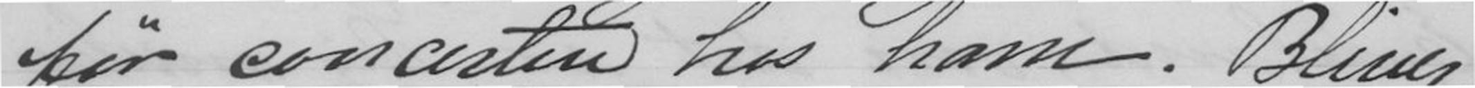før concerten hos ham. Bliver
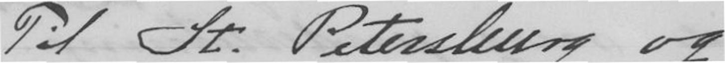Til St. Petersburg og
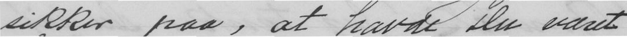sikker paa, at havde Du været
